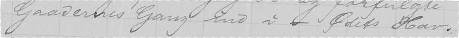Gaadernes Gang ind i - Ødets Hav.
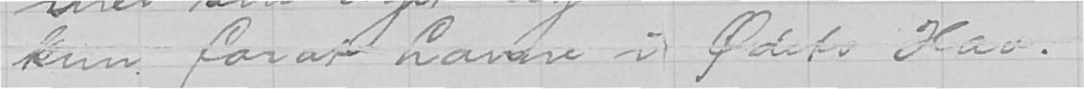kun forat havne i Ødets Hav.
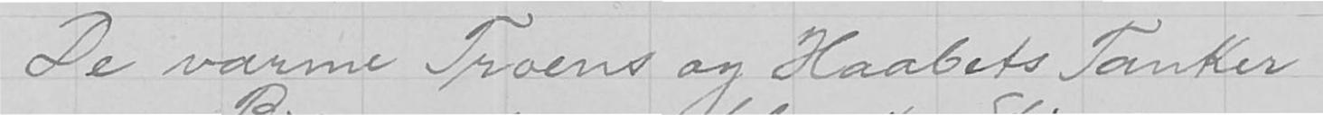De varme Troens og Haabets Tanker
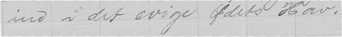ind i det evige Ødets Hav.
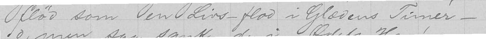flød som en Livs-flod i Glædens Timer -
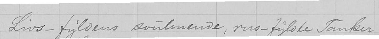Livs-fyldens svulmende, rus-fyldte Tanker
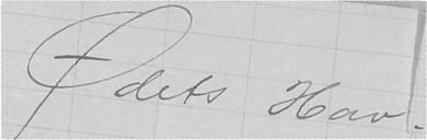Ødets Hav.
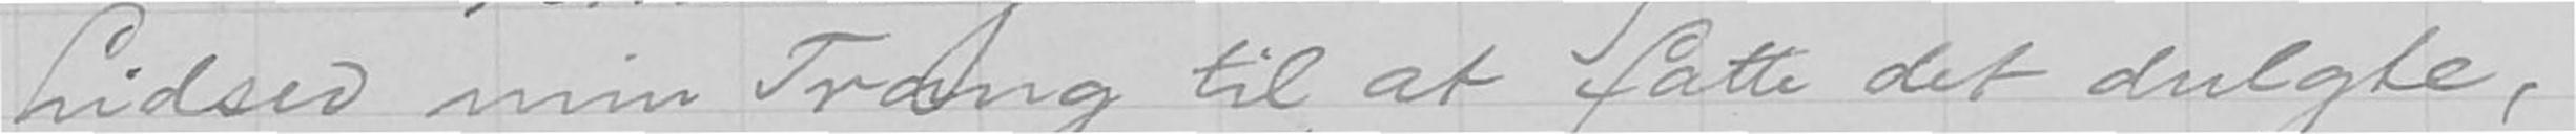hidser min Trang til at fatte det dulgte,
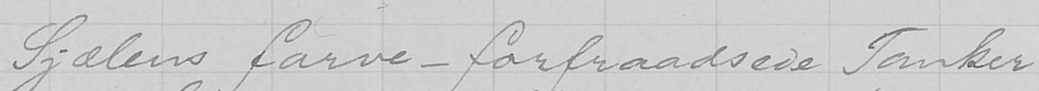Sjælens farve-forfraadsede Tanker
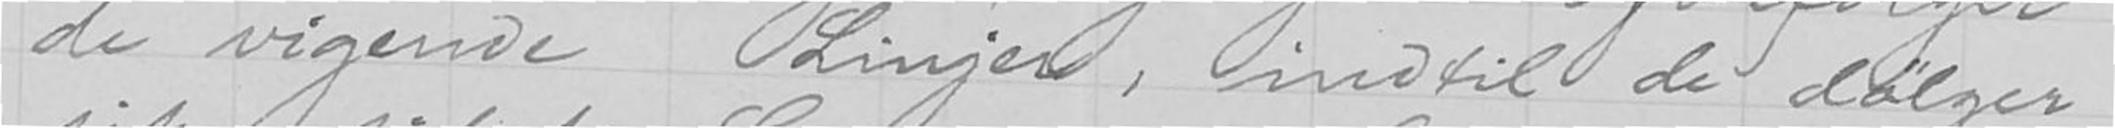de vigende Linjer, indtil de dølger
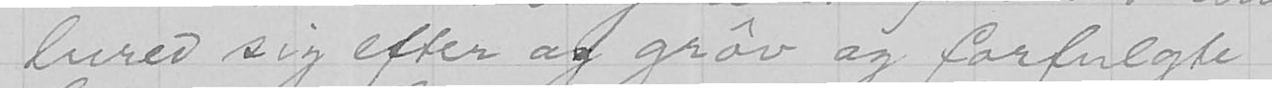lured sig efter og grôv og forfulgte
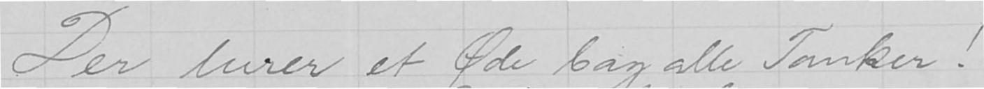Der lurer et Øde bag alle Tanker!
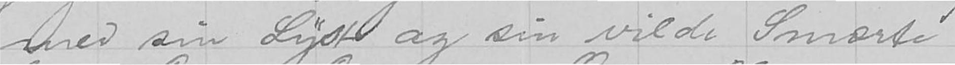med sin Lyst og sin vilde Smærte
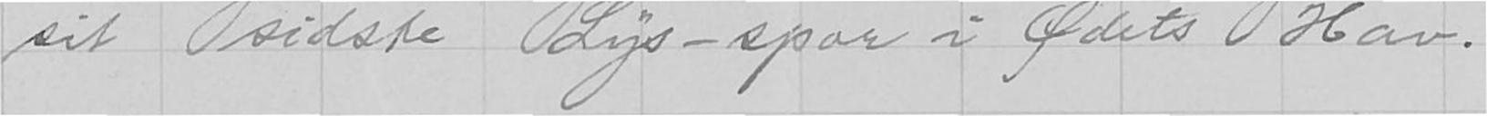sit sidste Lys-spor i Ødets Hav.
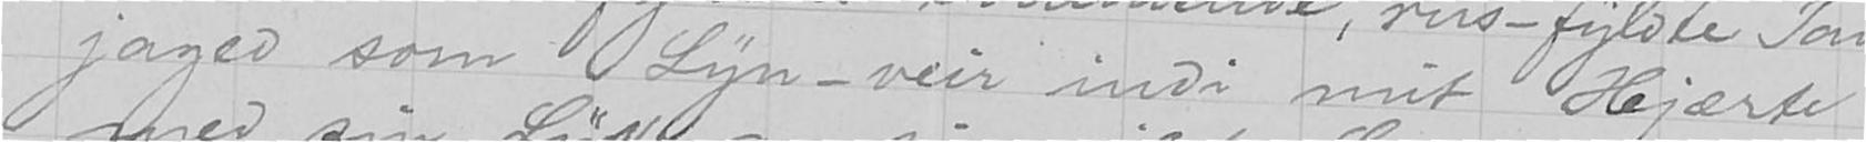jaged som Lyn-veir indi mit Hjærte
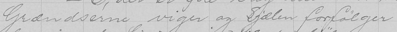Grændserne viger og Sjælen forfølger
